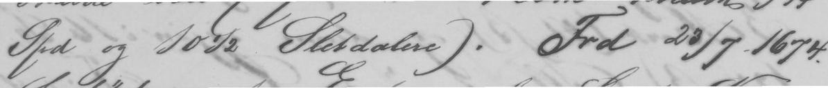Spd og 10½ Sletdalere). Frd 23/7 1674.
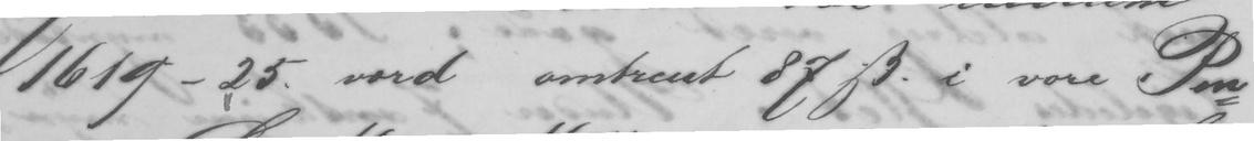1619-25 værd omtrent 87 ẞ.  i vore Pen-
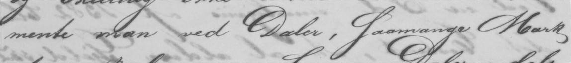mente man ved Daler, Saamange Mark
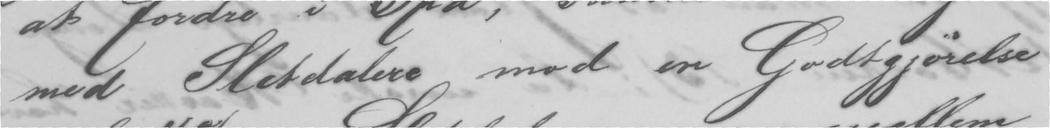med Sletdalere mod en Godtgjørelse
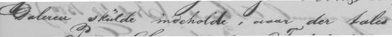Daleren skulde indeholde, naar der tales
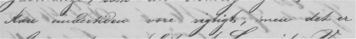kan undertiden være vigtigt, men det er
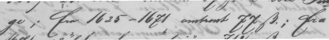ge; fra 1625-1671 omtrent 77 ẞ.; fra
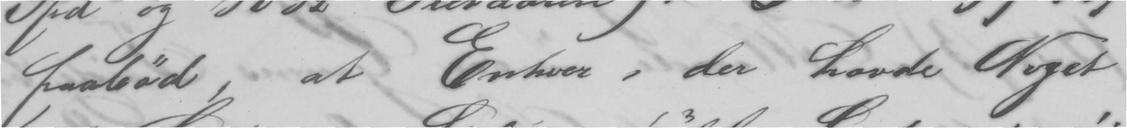frabød, at Enhver, der havde Noget
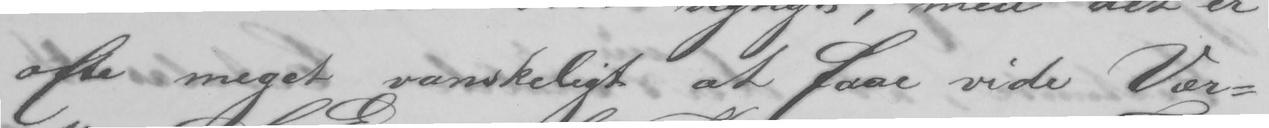ofte meget vanskeligt at faae vide Vær-
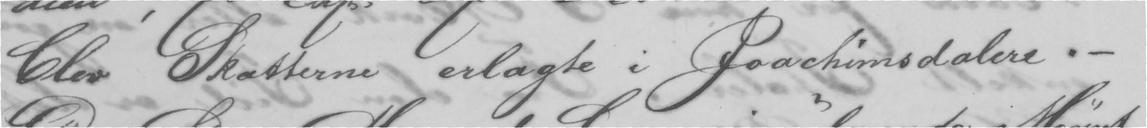blev Skatterne erlagte i Joachimsdalere. -
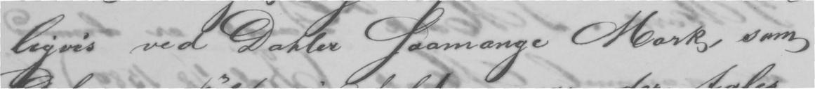ligvis ved Dahler Saamange Mark, som
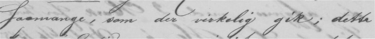Saamange, som der virkelig gik; i dette
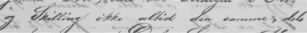og Skilling ikke altid den samme, dels
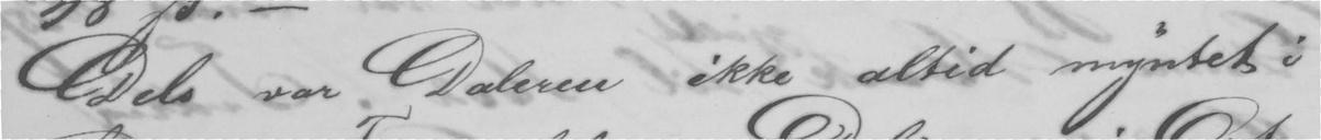Dels var Daleren ikke altid myntet i
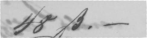48 ẞ. -
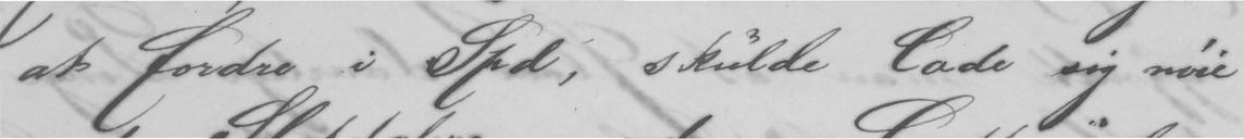at fordre i Spd, skulde lade sig nøie
